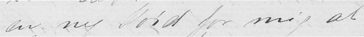en ny Jord for mig, at
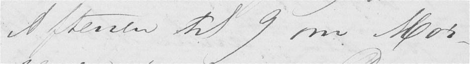Aftenen til 9 om Mor-
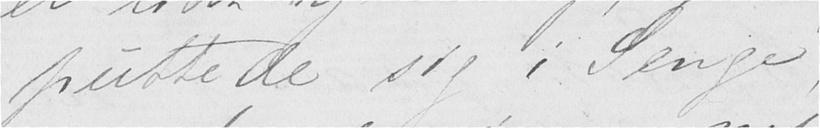puttede sig i Senge,
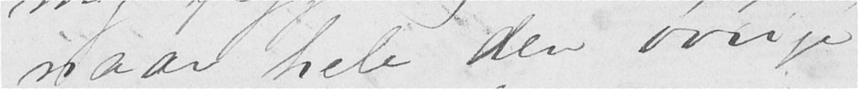naar hele den øvige
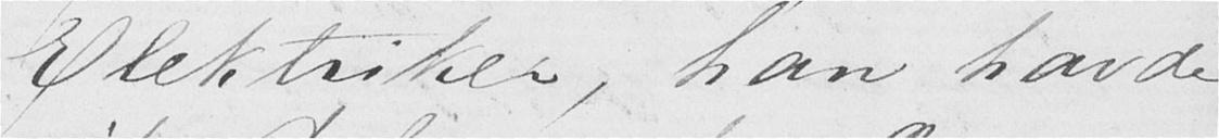Elektriker, han havde
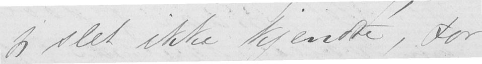jeg slet ikke kjendte, for
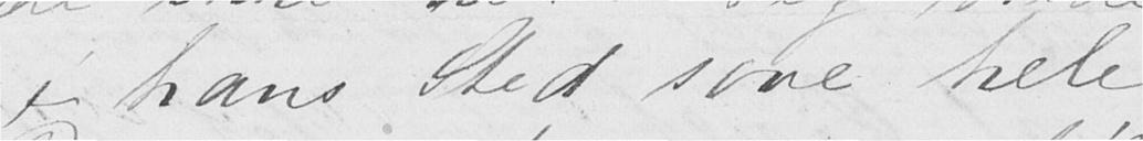i hans Sted sove hele
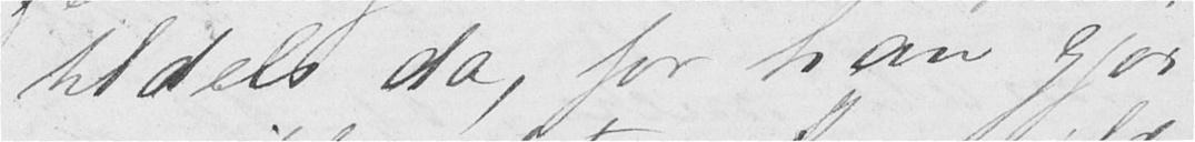tildels da, for han gjor-
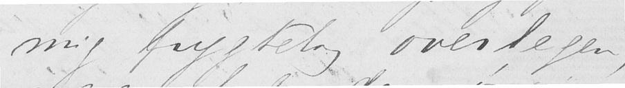mig frygtelig overlegen,
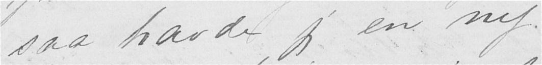saa havde jeg en ny
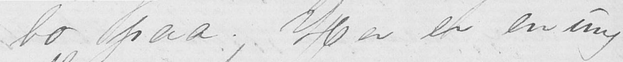bo paa. Her er en ung
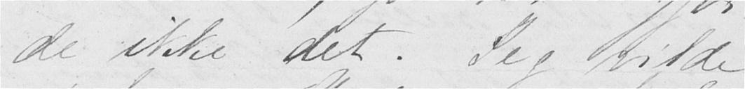de ikke det. Jeg vilde
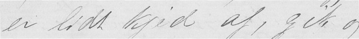er lidt kjed af, gik og
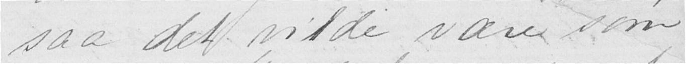saa det vilde være som
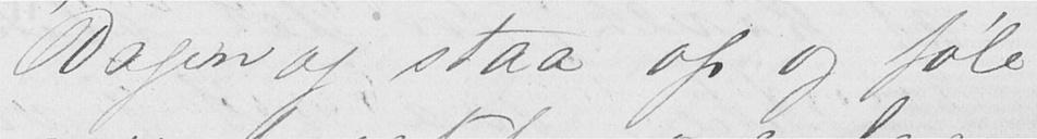Dagen og staa op og føle
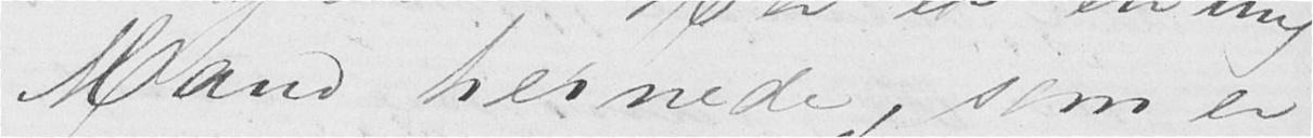Mand hernede, som er
